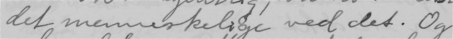det menneskelige ved det. Og
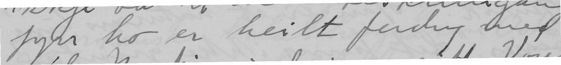fyrr ho er heilt ferdig med
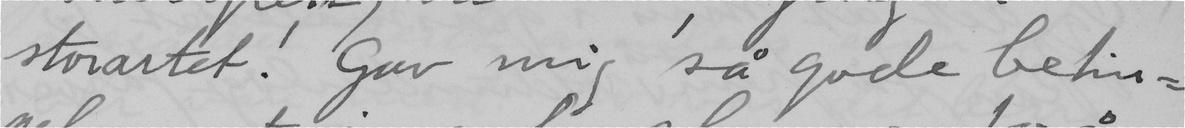storartet! Gav mig så gode betin-
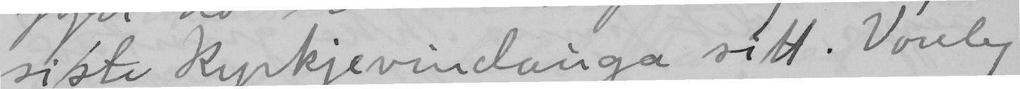siste kyrkjevindauga sitt. Vonleg
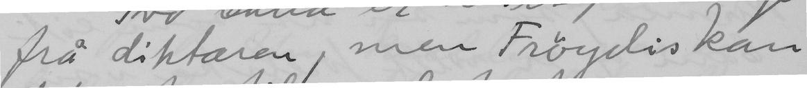frå diktaren, men Fröydis kan
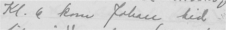Kl. 6 kom Johan hid
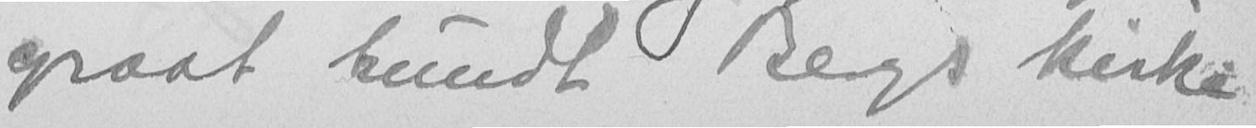graat kundt Bergs kirke
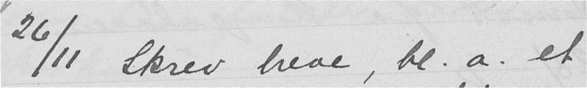26/11 Skrev breve, bl. a. et
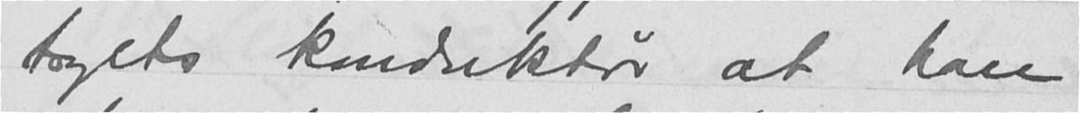togets konduktør at han
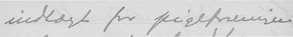indtægt for pigeforeningen
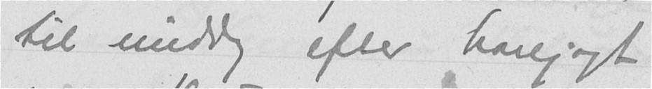til middag efter harejagt
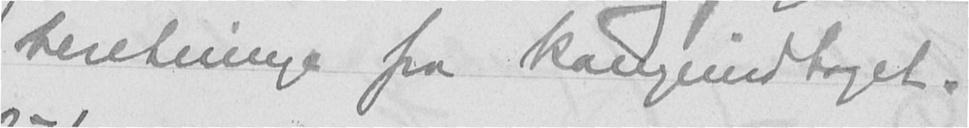beretninge fra kongeindtoget.
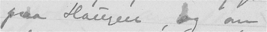paa Haugen, og om
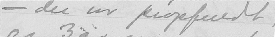- der var propfuldt,
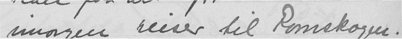imorgen reiser til Romskogen.
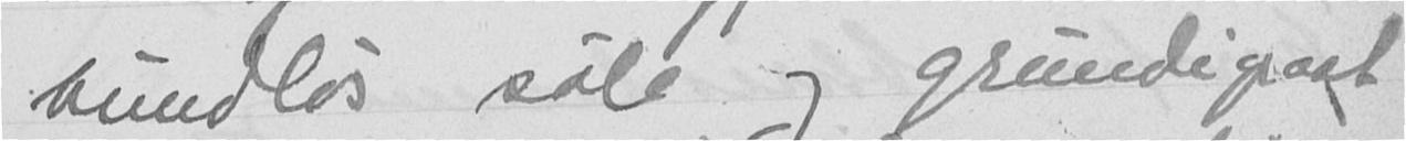bundløs søle og grundigaat
